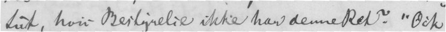tut, hvis Bestyrelse ikke har denne Ret? " Ock
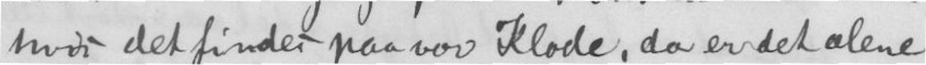hvis det finder paa vor Klode, da er det alene
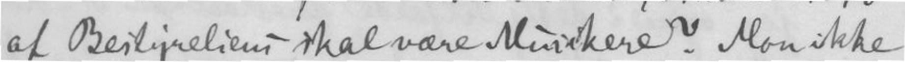af Bestyrelsens skal være Musikere? Mon ikke
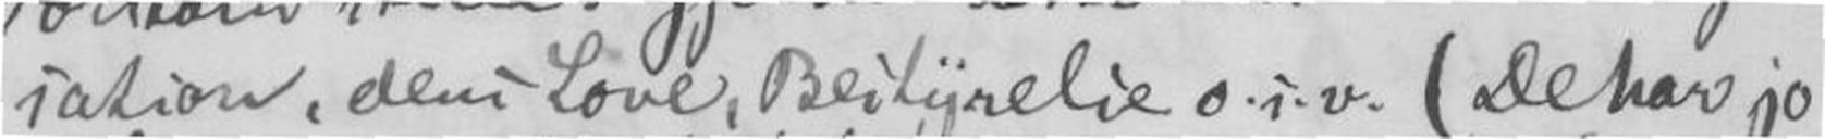sation, dem Love, Bestyrelse o.s.v. (De har jo
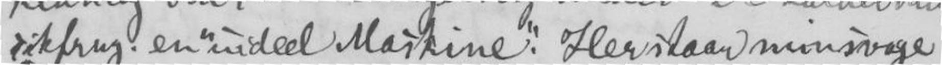sikfrng. en "uideel Maskine". Her staar min svage
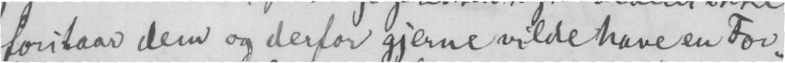forstaar dem og derfor gjerne vilde have en For-
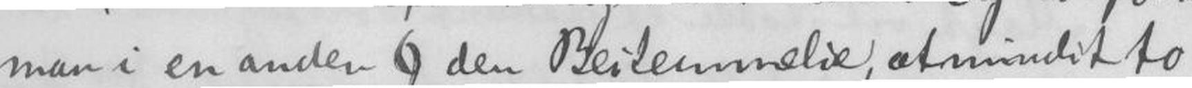man i en anden () den Bestemmelse, at mindst to
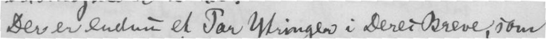Der er endnu et Par Ytringer i Deres Breve, som
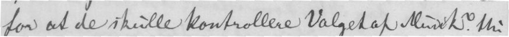for at de skulle kontrollere Valget af Musik? thi
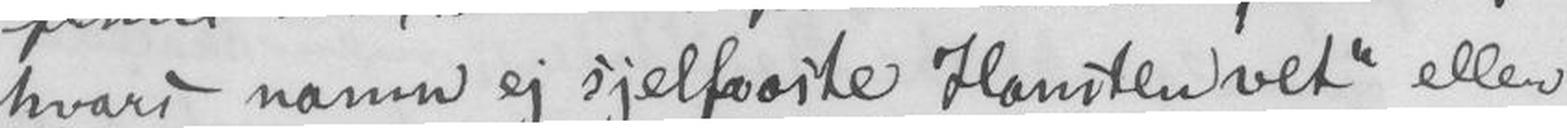hvari namn ej sjelfvæste Hansten ved" eller
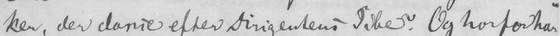ker, der Danse efter Dirigentens Pibe? Og hvorfor har
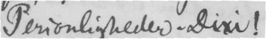Personligheder Deri!
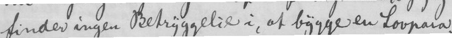finder ingen Betryggelse i, at bygge en Lovpa-
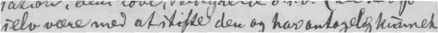selv være med at stifte den og har antagelig kunnet
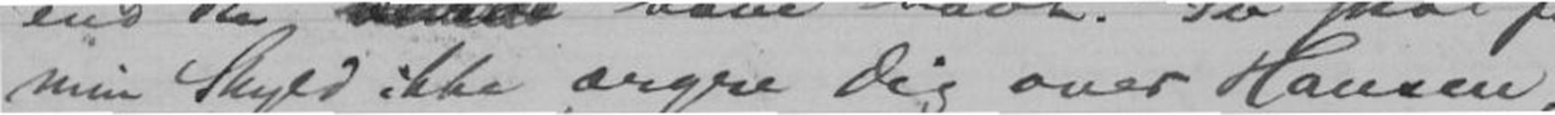min Skyld ikke ærgre Dig over Hansen,
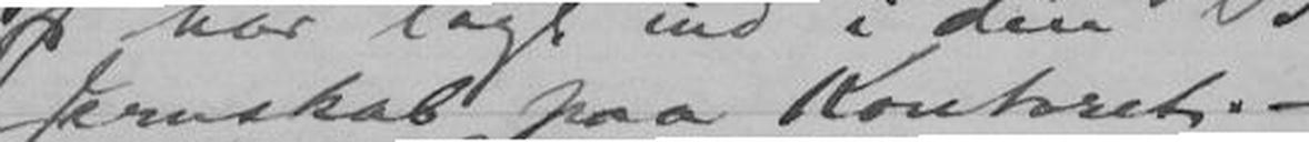Jernskab paa Kontoret. -
